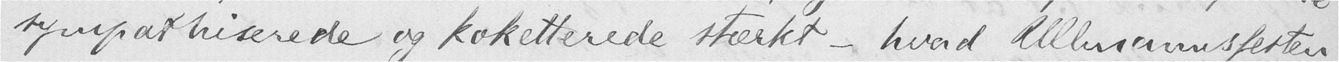sympathiserede og koketterede stærkt - hvad Ullmannsfesten
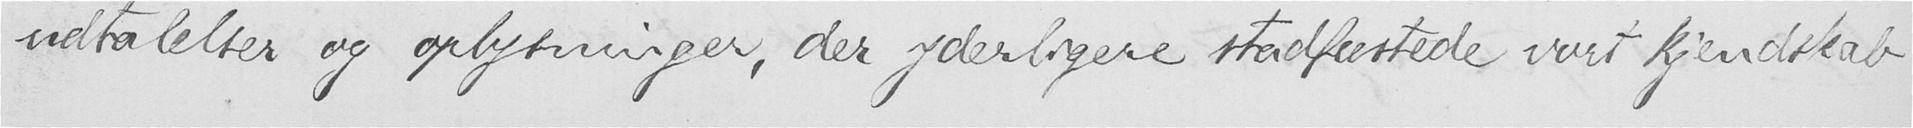udtalelser og oplysninger, der yderligere stadfæstede vort kjendskab
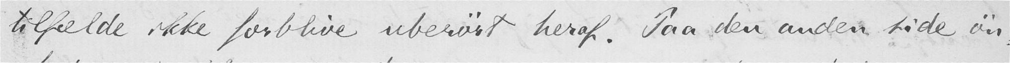tilfælde ikke forblive uberørt heraf. Paa den anden side øn-
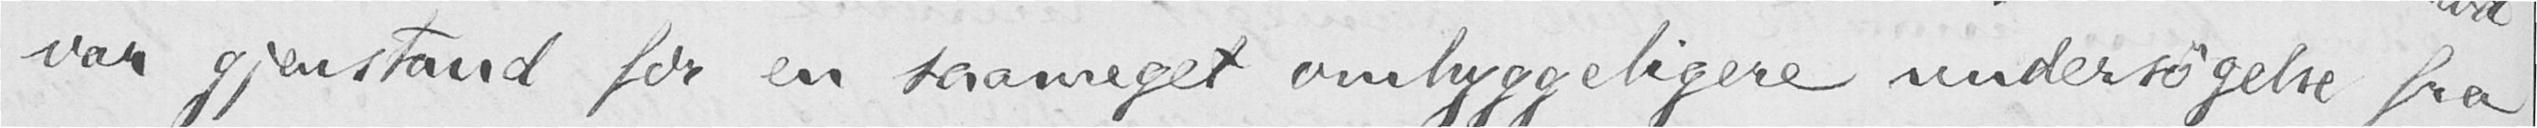var gjenstand for en saameget omhyggeligere undersøgelse fra
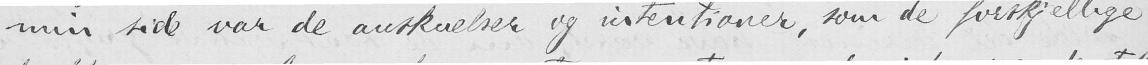min side var de anskuelser og intentioner, som de forskjellige
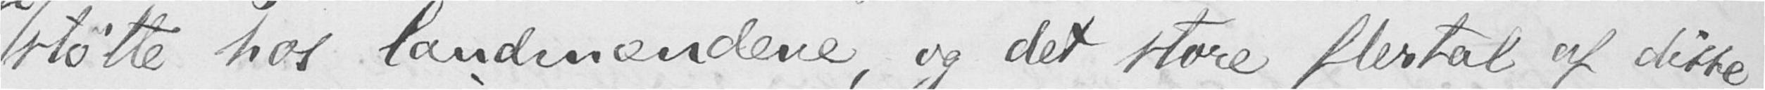støtte hos landmændene, og det store flertal af disse
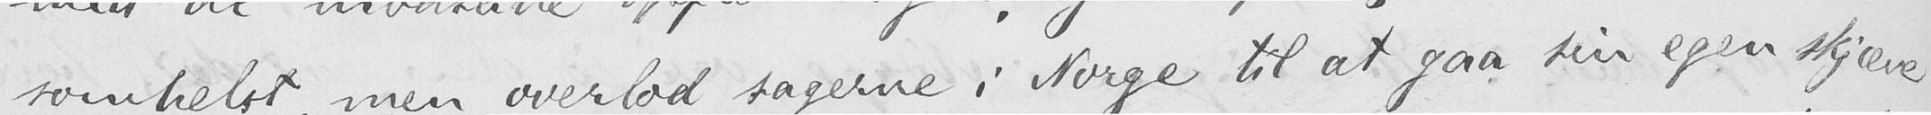somhelst, men overlod sagerne i Norge til at gaa sin egen skjæve
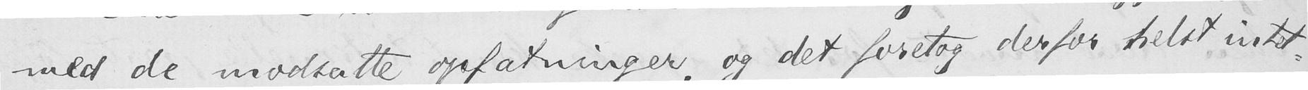med de modsatte opfatninger, og det foretog derfor helst intet-
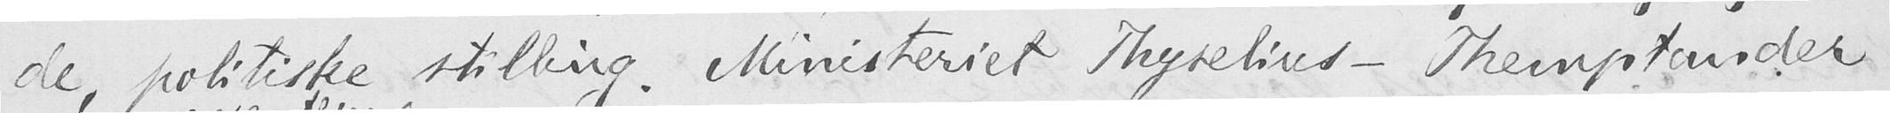de, politiske stilling. Ministeriet Thyselius-Themptander
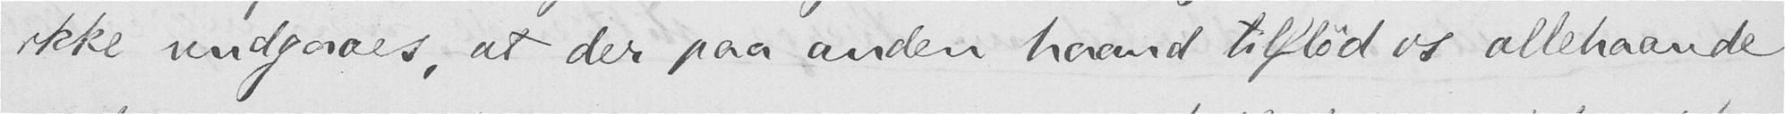ikke undgaaes, at der paa anden haand tilflød os allehaande
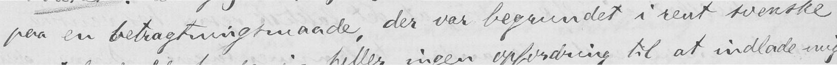paa en betragtningsmaade, der var begrundet i rent svenske
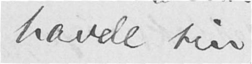havde sin
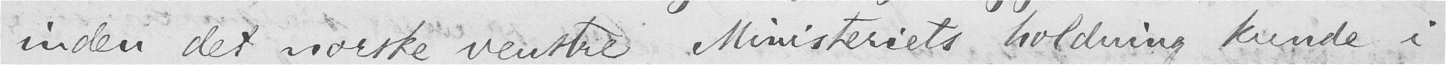inden det norske venstre. Ministeriets holdning kunde i
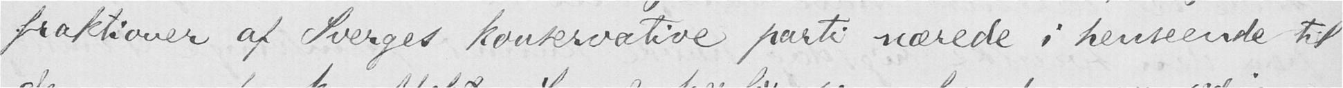fraktioner af Sverges konservative parti nærede i henseende til
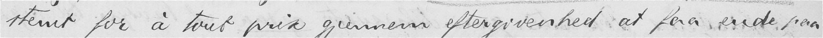stemt for à tout prix gjennem eftergivenhed at faa ende paa
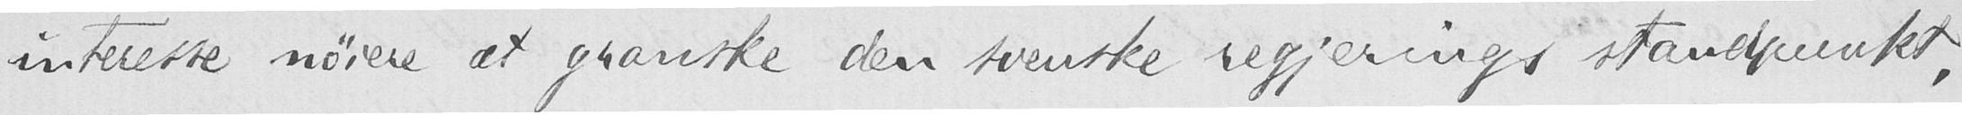interesse nøiere at granske den svenske regjerings standpunkt,
